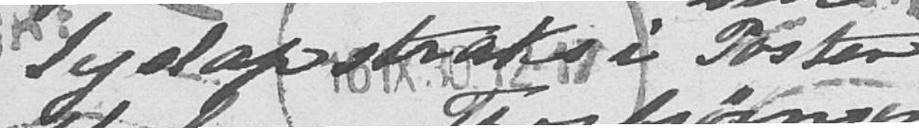Jeg slap straks i Posten
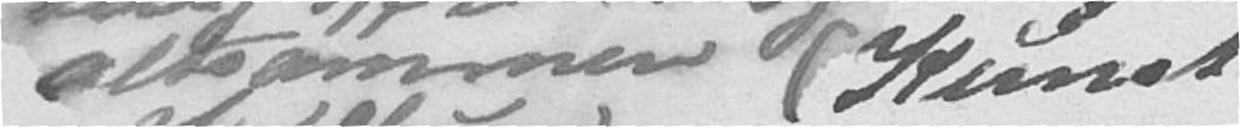altsammen (Kunst
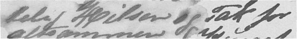telig Hilsen og Tak for
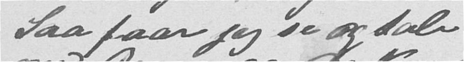Saa faar jeg se og tale
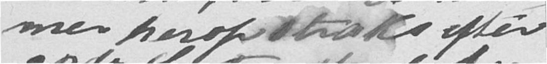mer herop straks efter
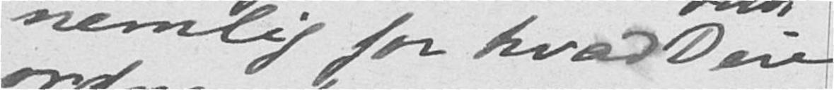nemlig for hvad Dere
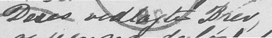Deres vedlagte Brev,
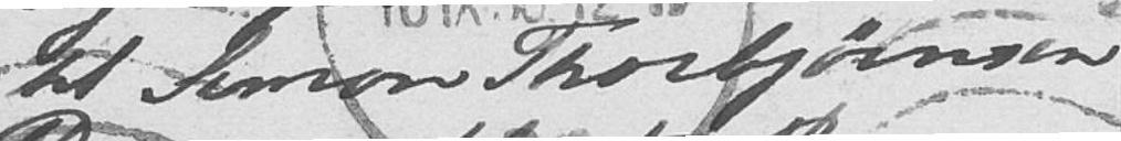til Simon Thorbjørnsen
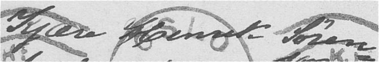Kjære Henrik Søren-
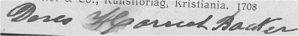Deres Harriet Backer
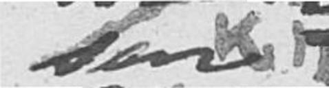sen
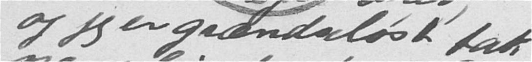og jeg er grændseløst tak
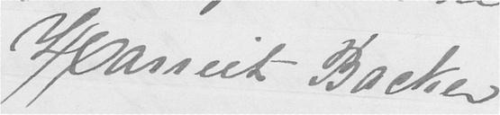Harriet Backer
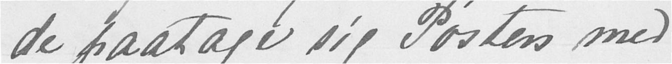de paatage sig Posten med
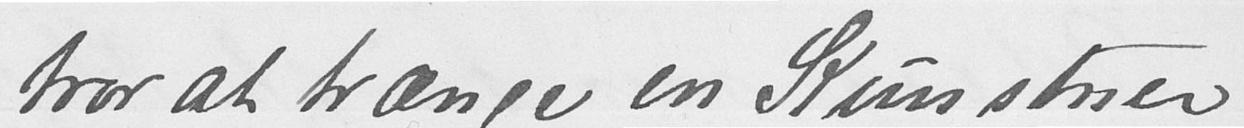tror at trænge en Kunstner
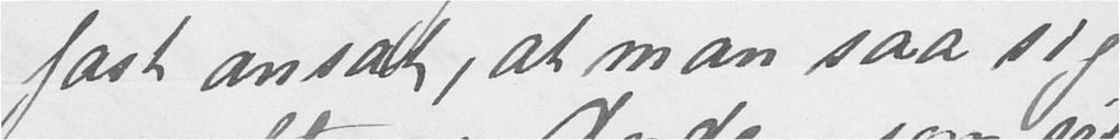fast ansat, at man saa sig
